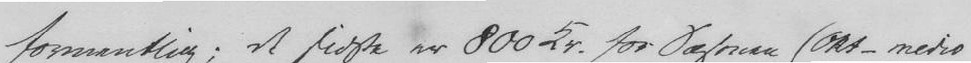formentlig; de sidste er 800 Kr. for Sæsonen (Okt-medio
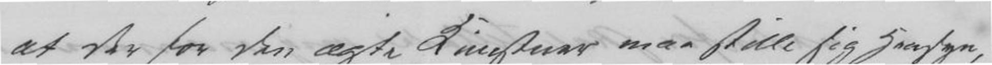at der for den ægte Kunstner maa stille sig Hensyn,
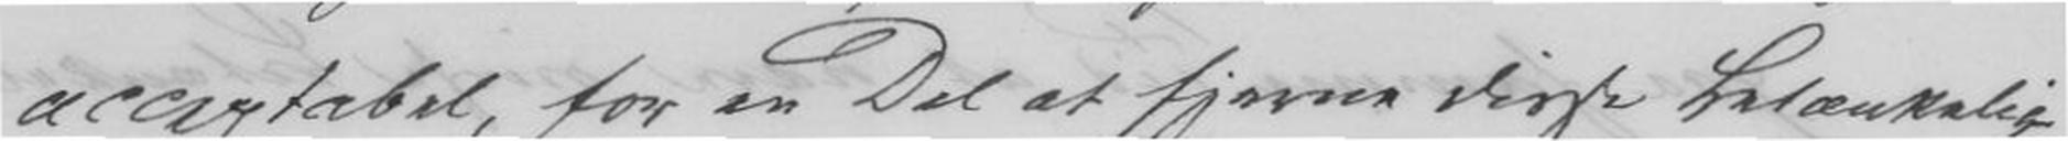acceptabel, for en Del at fjerne disse Betænkelig-
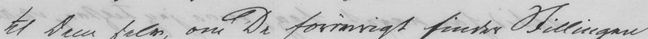til Dem selv, om De forøvrigt finder Stillingen
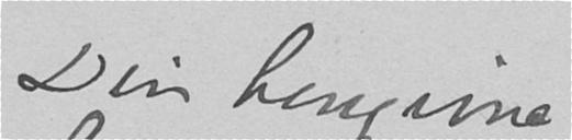Din hengivne
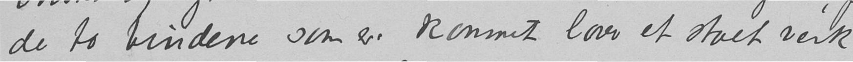de to bindene som er kommet lover et stort verk,
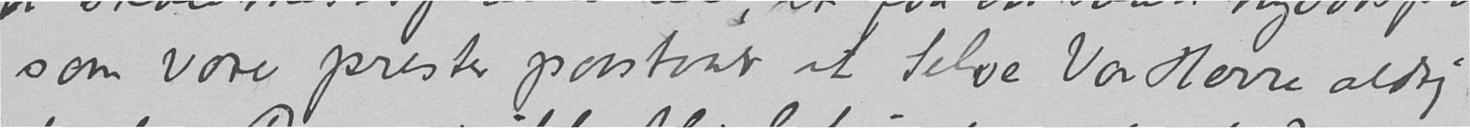- som vore prester paastaar at Selve Vor Herre aldrig
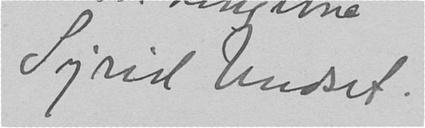Sigrid Undset.
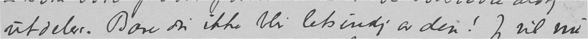utdeler. Bare du ikke blir letsindig av den! Jeg vil nu
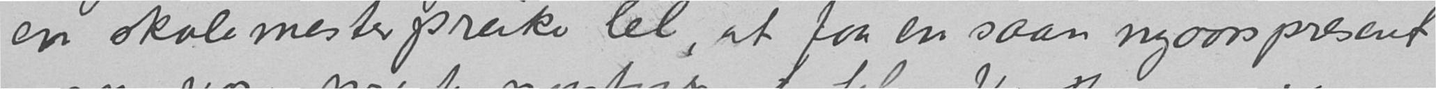en skolemesterpreike lel, at faa en saan nyaarspresent
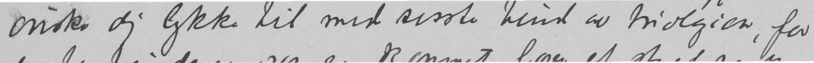ønske dig lykke til med sisste bind av triologien, for
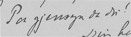Paa gjensyn da du!
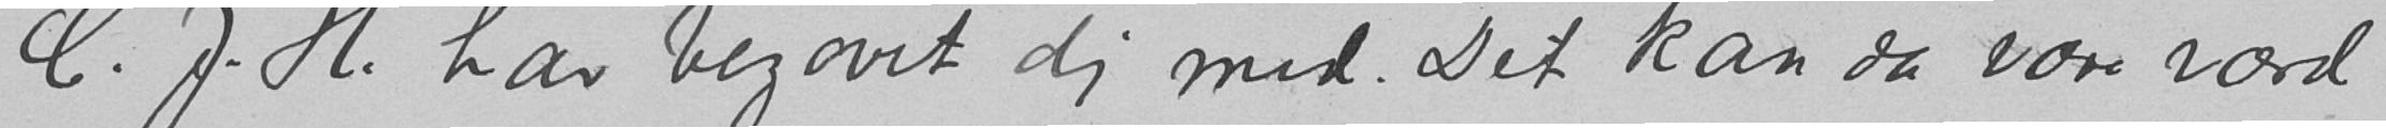C.J.H. har begavet dig med. Det kan da være værd
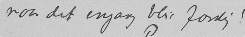naar det engang blir færdig!
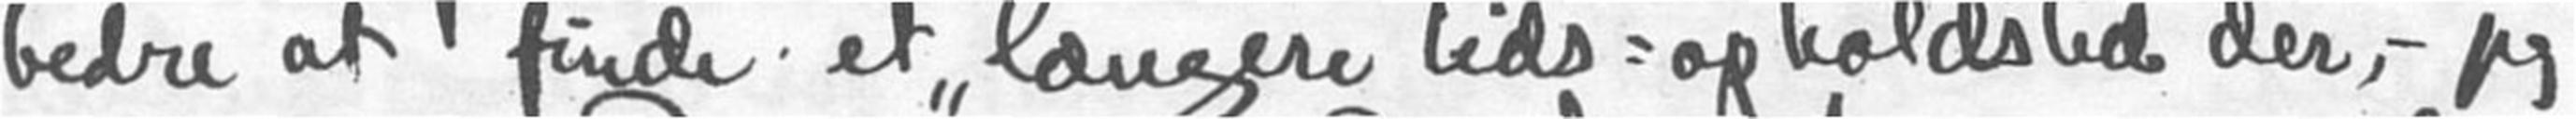bedre at finde et "længere tids-opholdsted" der, - jeg
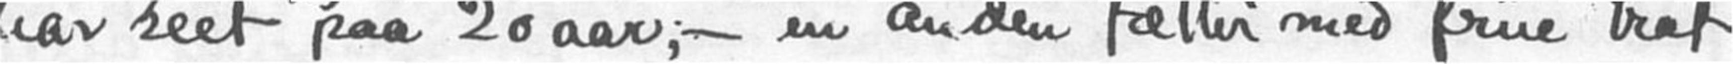har seet paa 20 aar, - en anden fetter med frue traf
